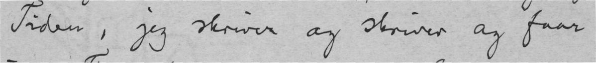Tiden, jeg skriver og skriver og faar
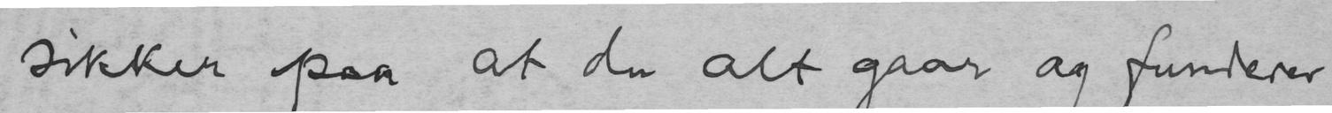sikker paa at du alt gaar og funderer
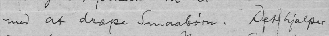med at dræpe Smaabørn. Det hjælper
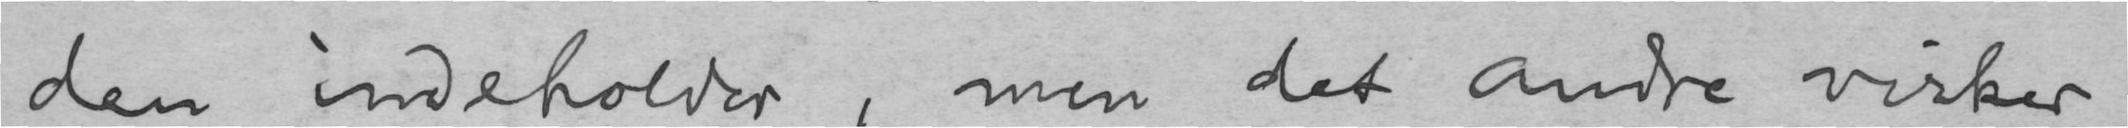den indeholder, men det andre virker
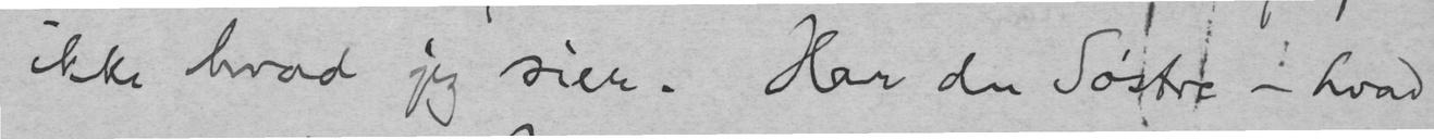ikke hvad jeg sier. Har du Søstre - hvad
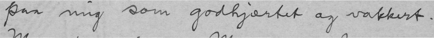paa mig som godhjærtet og vakkert.
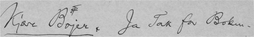Kjære Bojer. Ja Tak for Boken.
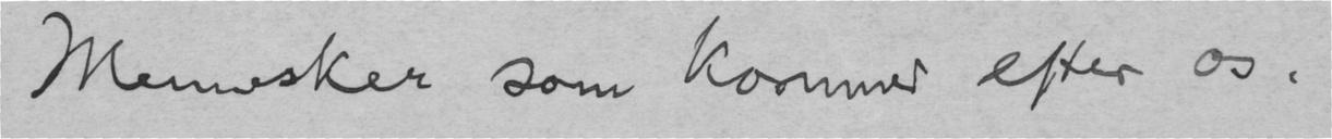Mennesker som kommer efter os.
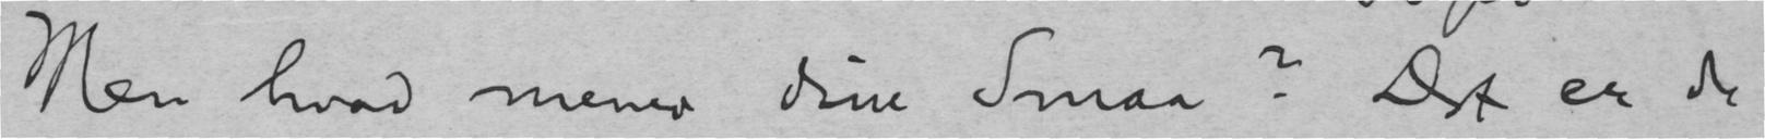Men hvad mener dine Smaa? Det er de
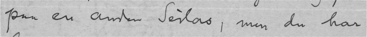paa en anden Seilas, men du har
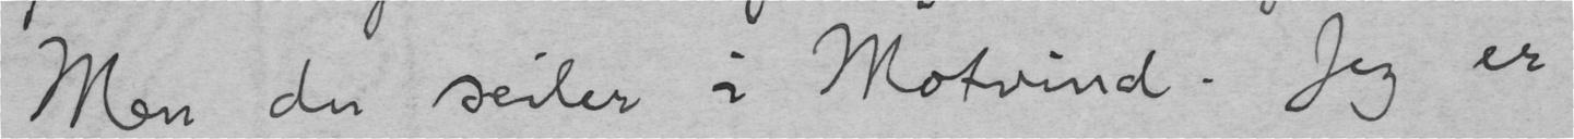Men du seiler i Motvind. Jeg er
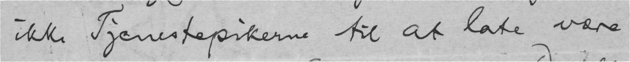ikke Tjenestepikerne til at late være
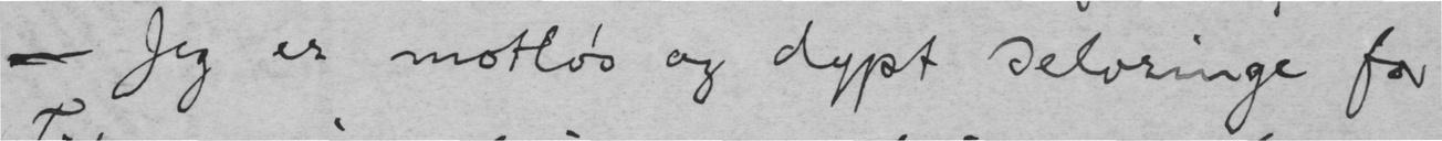- Jeg er motløs og dypt selvringe for
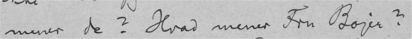mener de? Hvad mener Fru Bojer?
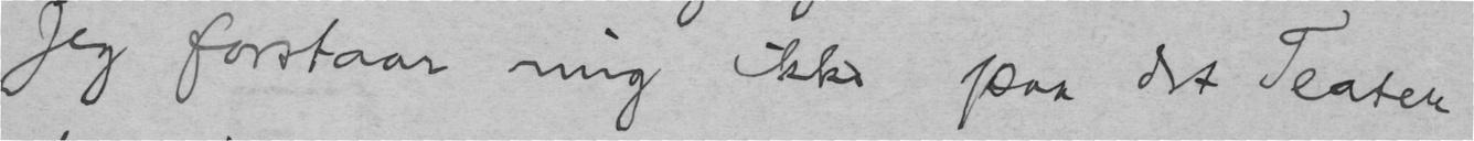Jeg forstaar mig ikke paa det Teater
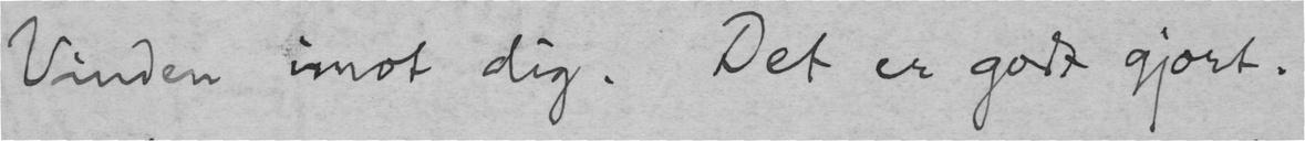Vinden imot dig. Det er godt gjort.
